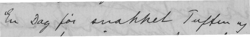En Dag før snakket Tuften og
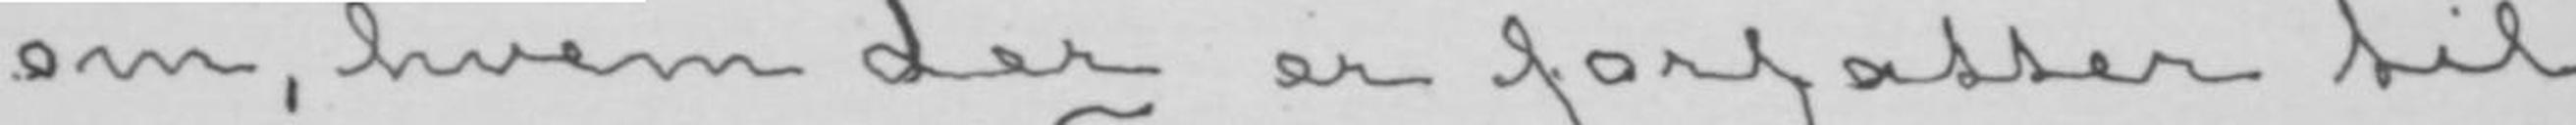om, hvem der er forfatter til
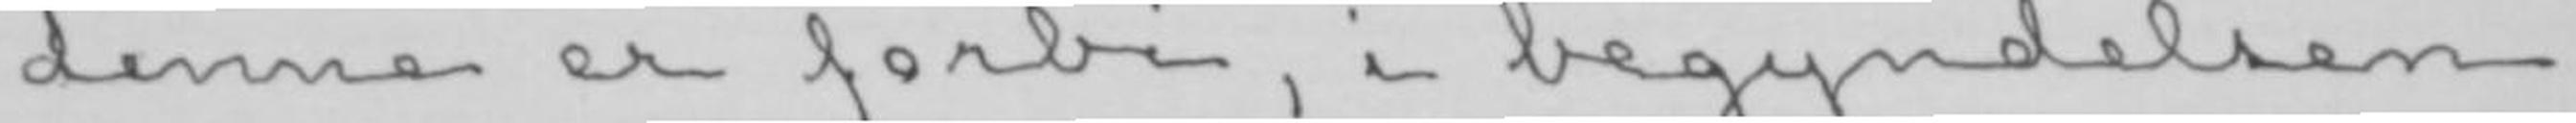denne er forbi, i begyndelsen
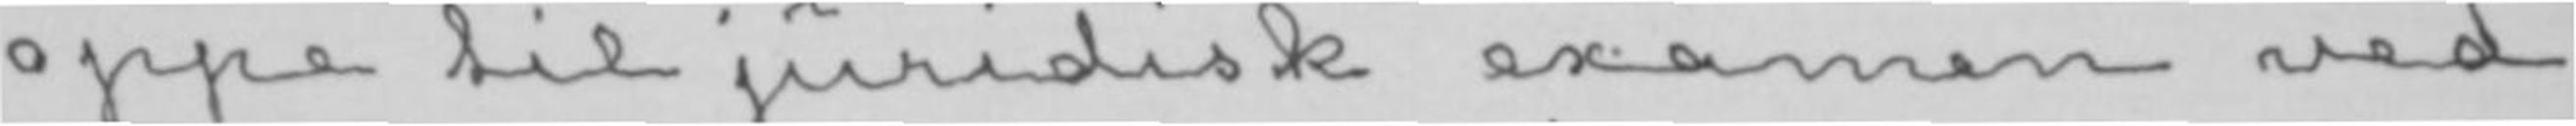oppe til juridisk examen ved
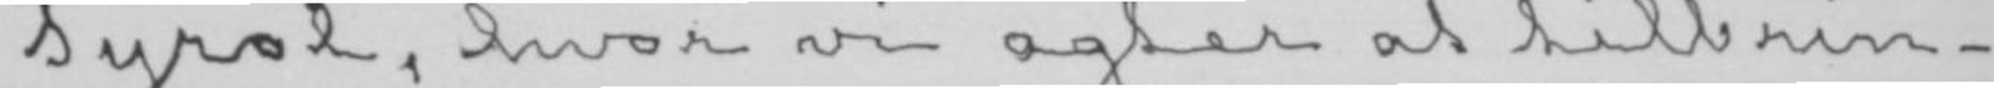Tyrol, hvor vi agter at tilbrin-
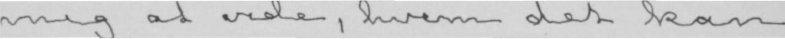mig at vide, hvem det kan
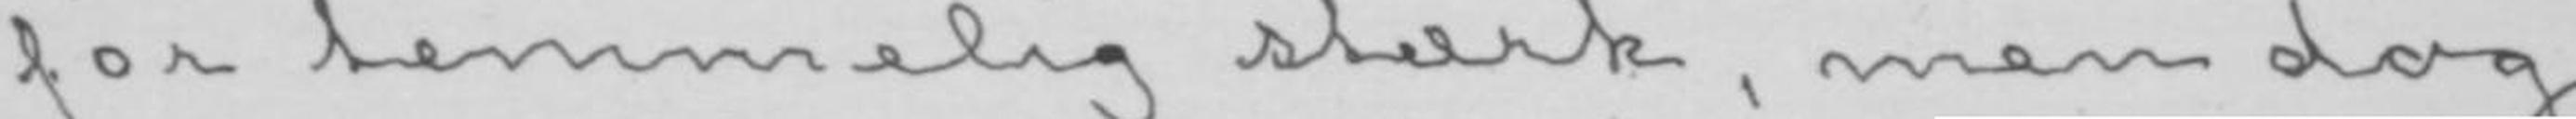for temmelig stærk, men dog
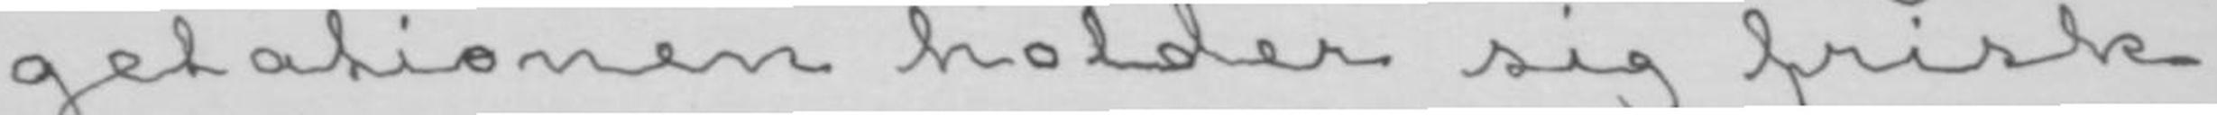getationen holder sig frisk
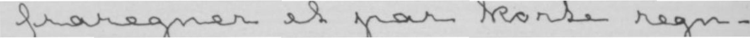jeg fraregner et par korte regn-
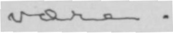være.
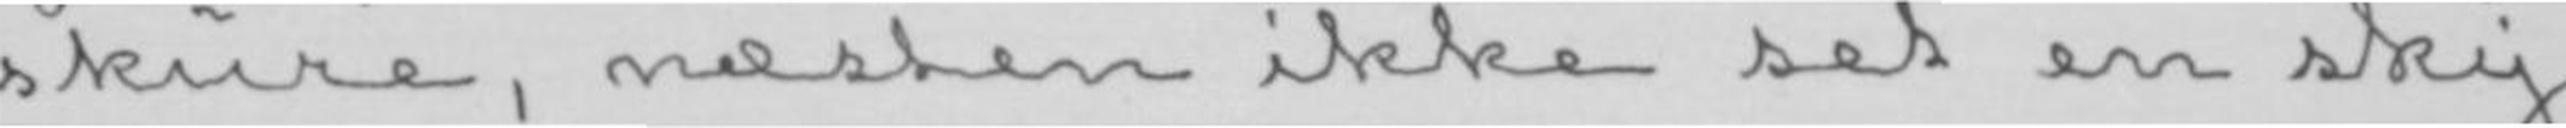skure, næsten ikke set en sky
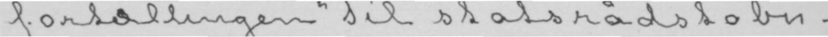fortællingen «Til statsrådstabu-
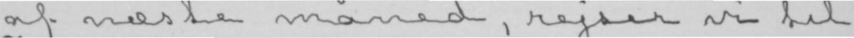af næste måned, rejser vi til
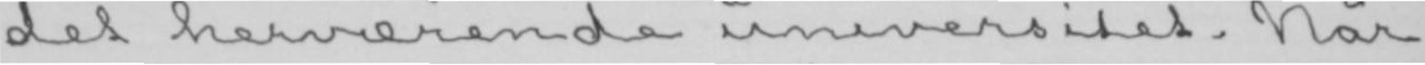det herværende universitet. Når
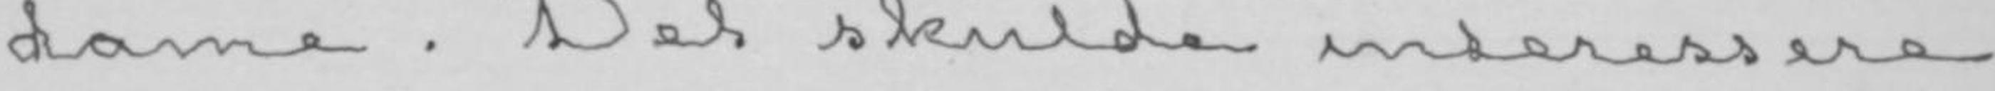dame. Det skulde interessere
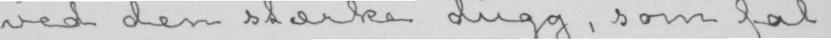ved den stærke dugg, som fal-
